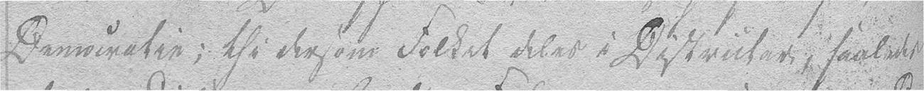Democratie; thi dersom Folket deles i Districter, saaledes
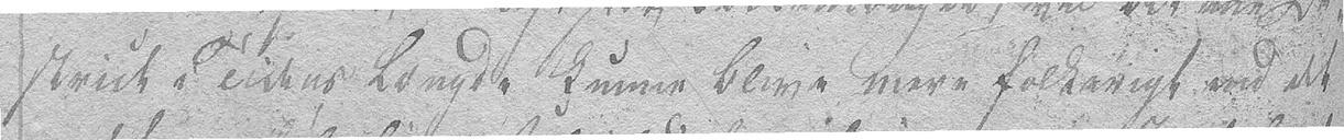strict i Tidens Længde kunne blive mere folkerigt end det
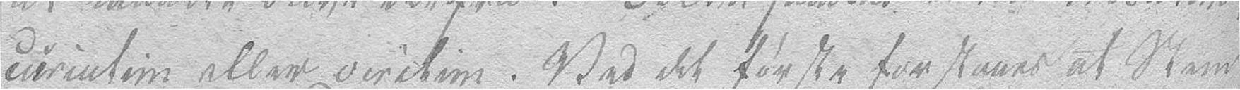Curintion eller Directim. Ved det første forstaaes at Stem-
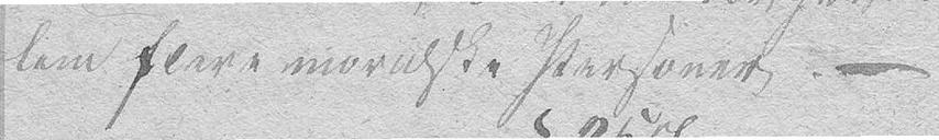lem flere moralske Personer. –
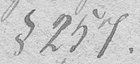§ 257.
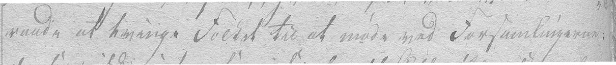raade af tvinge Folket til at møde ved Forsamlingerne:
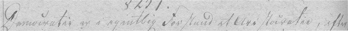Democratie er i egentlig Forstand et Aristocratie, efter-
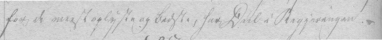for de meest oplyste og bedste, har Deel i Regjeringen. –
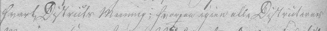hvert Districts Mening; hvorpaa igjen alle Districternes
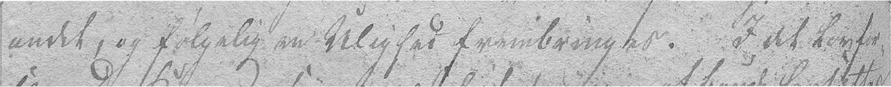andet, og følgelig en Ulighed frembringes. I det Lovfore-
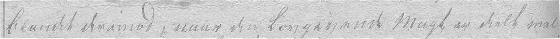blandet derimod, naar den Lovgivende Magt er deelt mel-
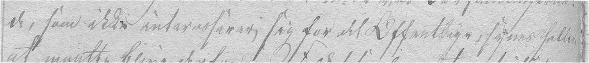de som ikke interesserer sig for det Offentlige synes heller
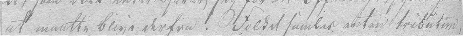at maatte blive derfra. Folket samles enten tributum
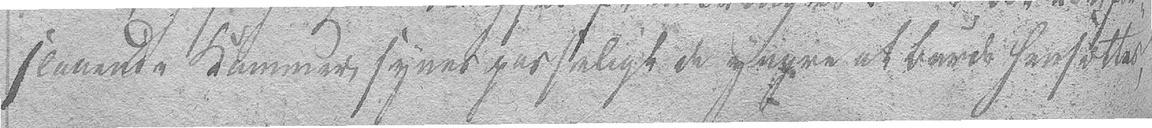slaaende Kammer synes passeligt de yngre at burde hensættes,
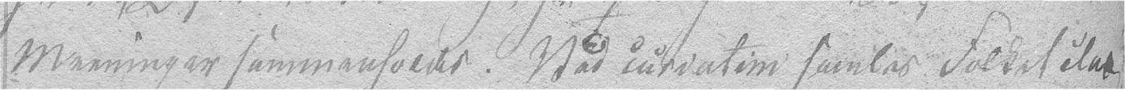Meninger sammenholdes. Ved curatim samles Folket clas-
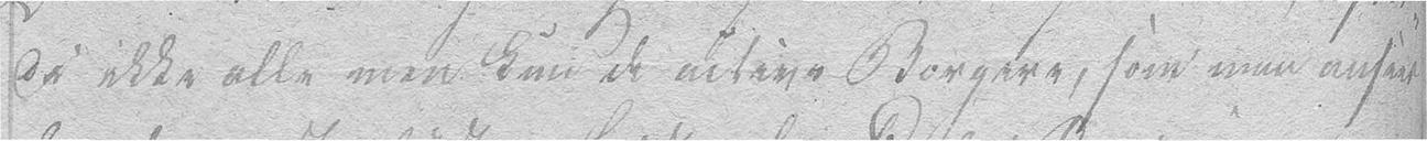di ikke alle men kun de active Borgere, som man anseer
